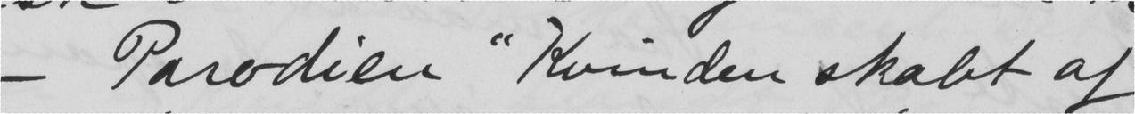- Parodien "Kvinden skabt af
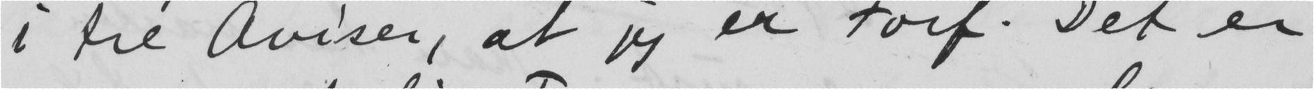i tre aviser, at jeg er Forf. Det er
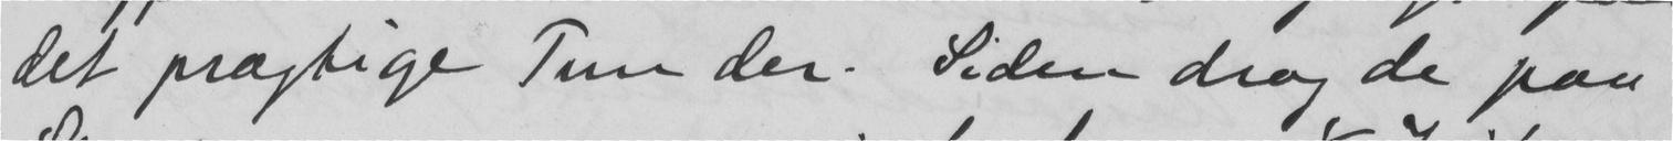det pragtige Tun der. Siden drog de paa
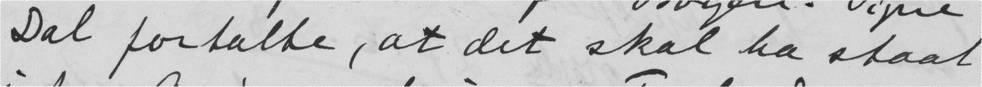Dal fortalte, at det skal ha staat
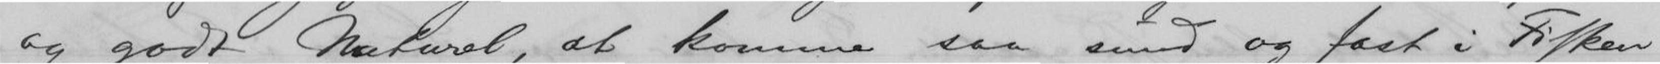og godt Naturel, at komme saa sund og fast i Fisken
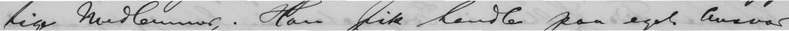tige Medlemmer. Han fik handle paa eget Ansvar
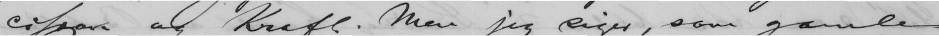cision og Kraft. Men jeg siger, som gamle
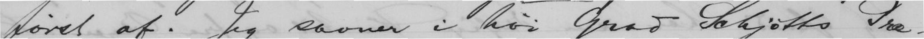først af. Jeg savner i høi Grad Schjøtts Præ-
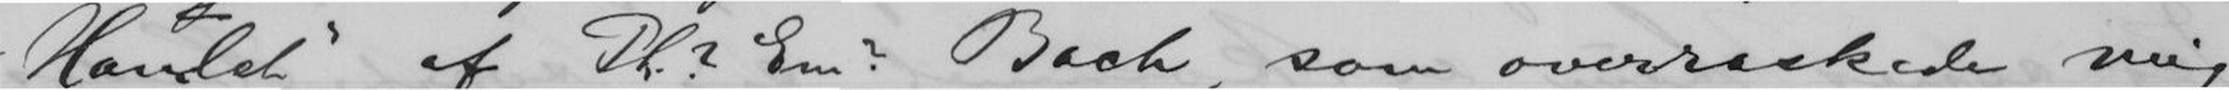"Hamlet" af Ph? Em? Bach, som overraskede mig
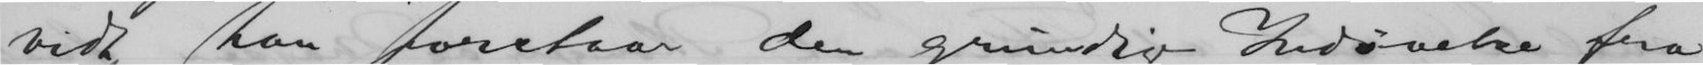vidt han forstaar den grundige Indøvelse fra
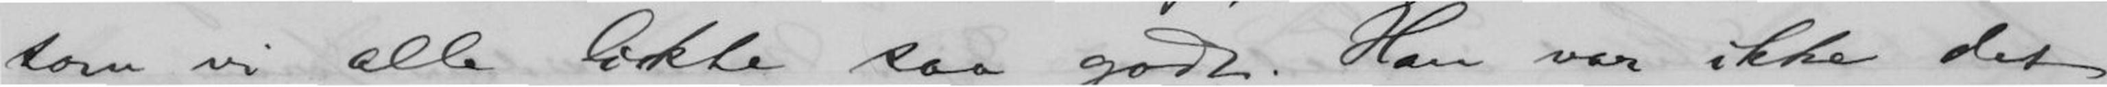som vi alle likte saa godt. Han var ikke det
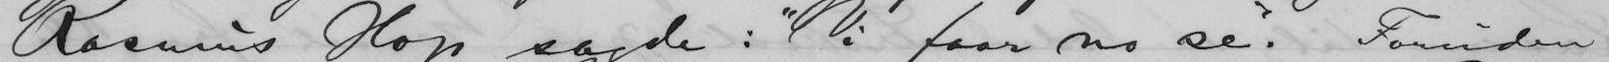Rasmus Hop sagde: "Vi faar no se". Foruden
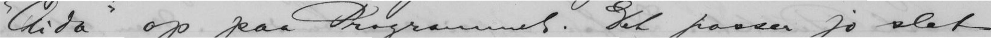"Aida" op paa Programmet. Det passer jo slet
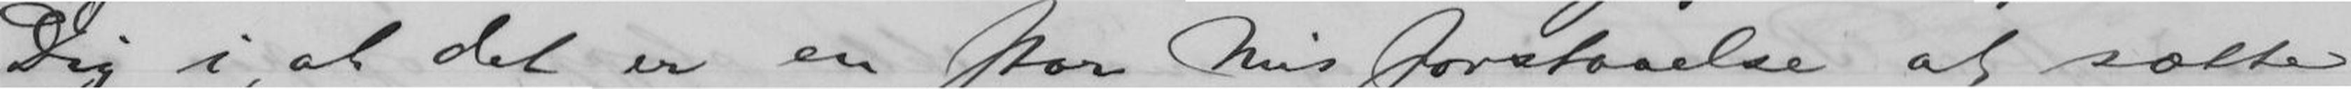Dig i, at det er en stor Misforstaaelse at sætte
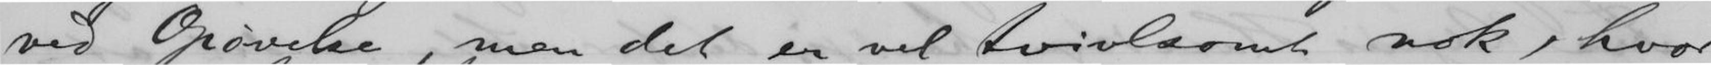ved Opøvelse, men det er vel tvivlsomt nok, hvor-
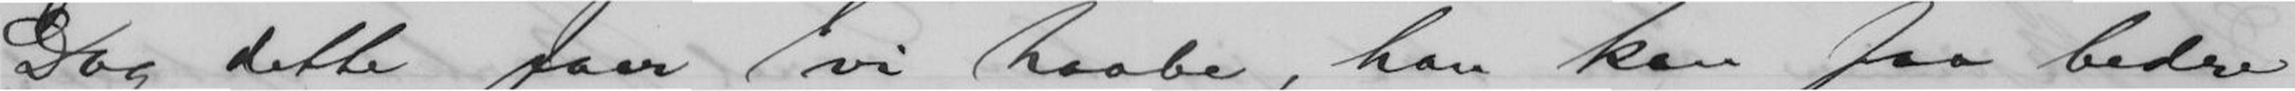Dog dette faar vi haabe, han kan faa bedre
